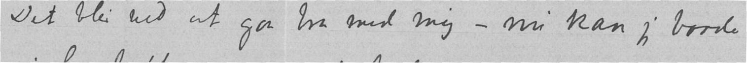Det blir ved at gaa bra med mig - nu kan jeg baade
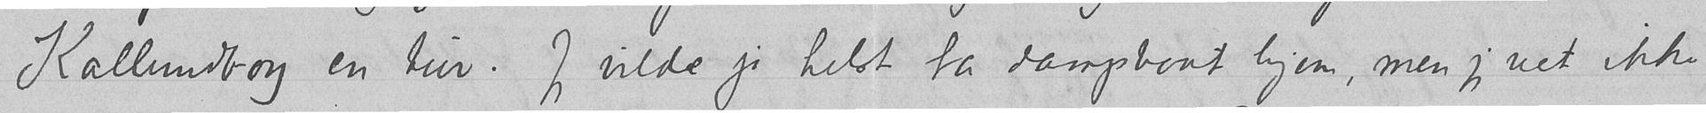Kallundborg en tur. Jeg vilde jo helst ta dampbaat hjem, men jeg vet ikke
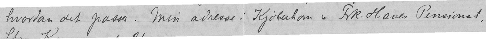hvordan det passer. Min adresse i Kjøbenhavn er Frk. Haves Pensionat,
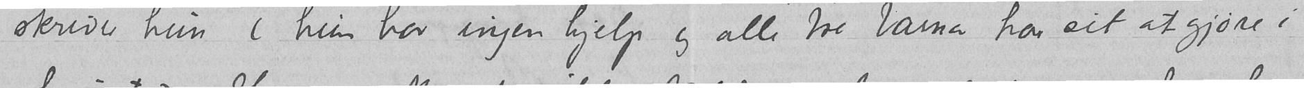skriver hun (hun har ingen hjelp og alle tre barna har sit at gjøre i
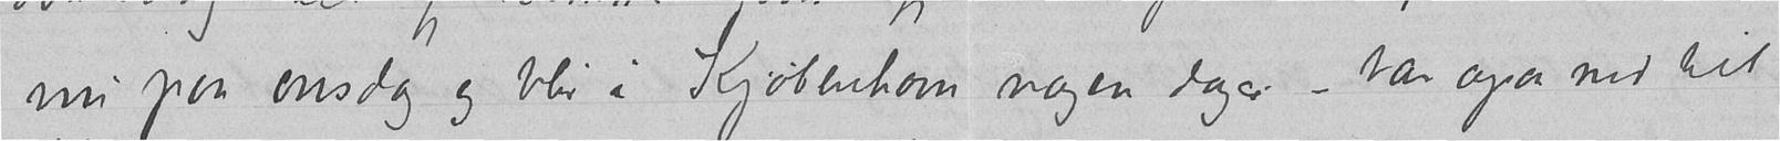nu paa onsdag og blir i Kjøbenhavn nogen dager – tar ogsaa ned til
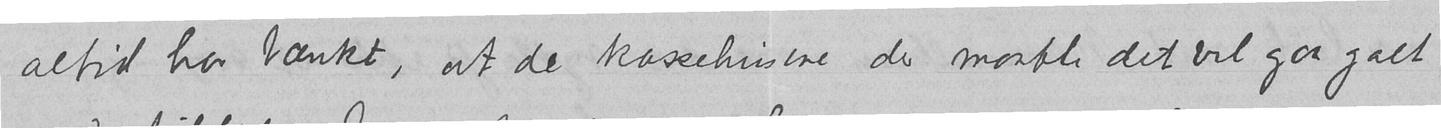altid har tænkt, at de kassehusene der maatte det vel gaa galt
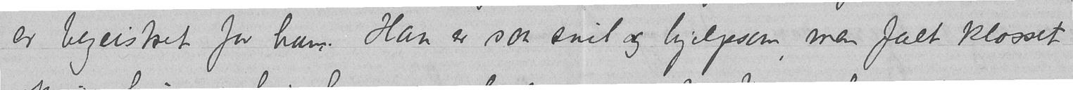er begeistret for ham. Han er saa snil og hjelpsom, men fælt klosset
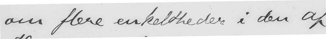om flere enkeltheder i den af
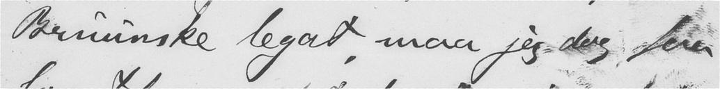Bruunske legat, maa jeg dog faa
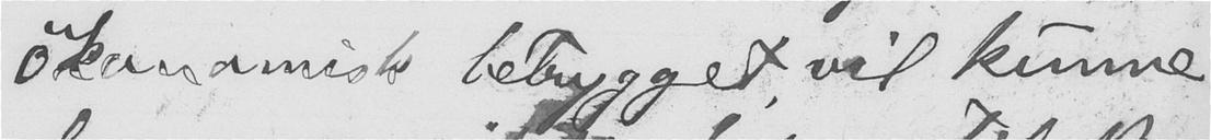økonomisk betrygget vil kunne
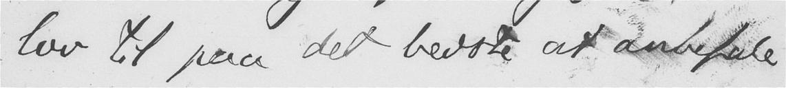lov til paa det bedste at anbefale
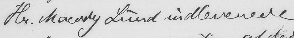Hr. Macody Lund indleverede
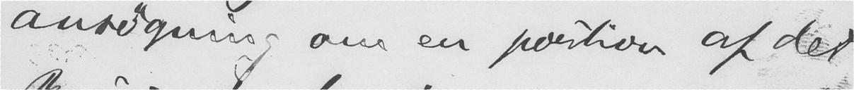ansøgning om en portion af det
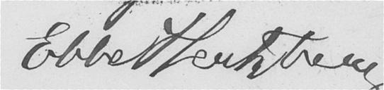Ebbe Hertzberg
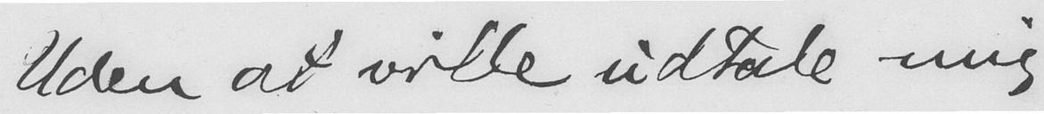Uden at ville udtale mig
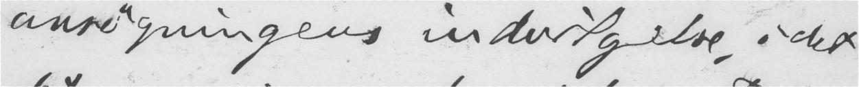ansøgningens indvilgelse, idet
